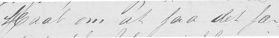Haab om at faa det fær-
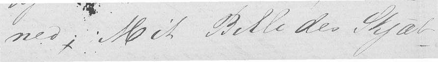ned. Mit Billedes Skjæb-
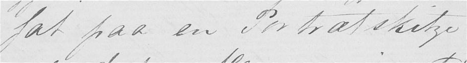fat paa en Portrætskitze
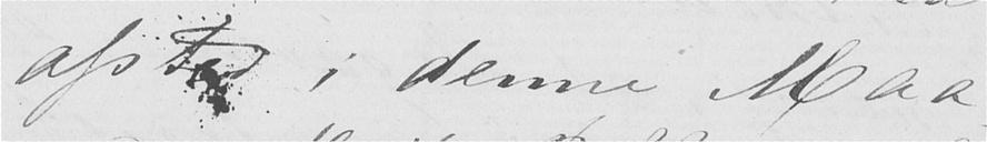afsted i denne Maa-
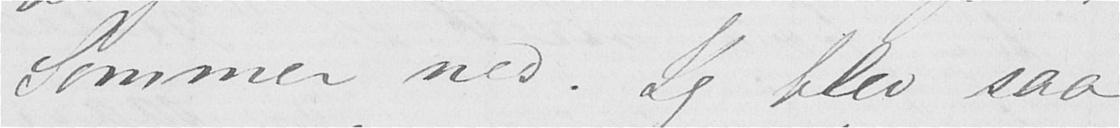Sommer ned. Jeg blev saa
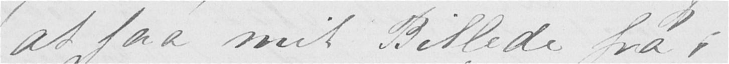at faa mit Billede fra i
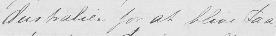Australien for at blive Faa-
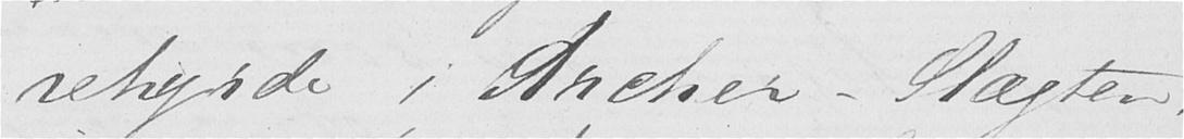rehyrde i Archer-Slægten.
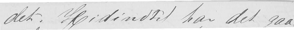det. Hidindtil har det gaa-
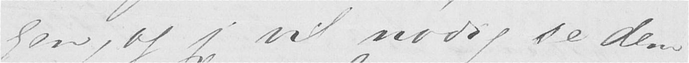gen, og jeg vil nødig se dem
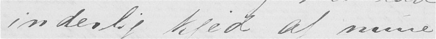inderlig kjed af mine
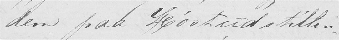dem paa Høstudstillin-
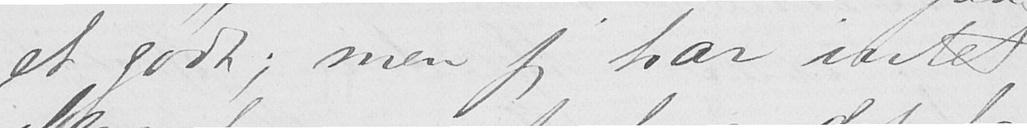et godt; men jeg har intet
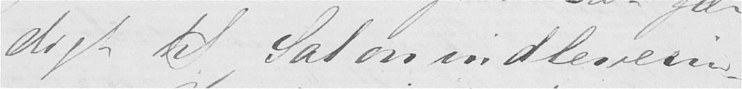digt til Salonindleverin-
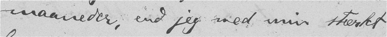maaneder, end jeg med min stærkt
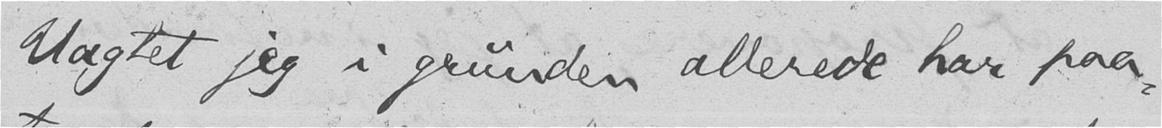Uagtet jeg i grunden allerede har paa-
